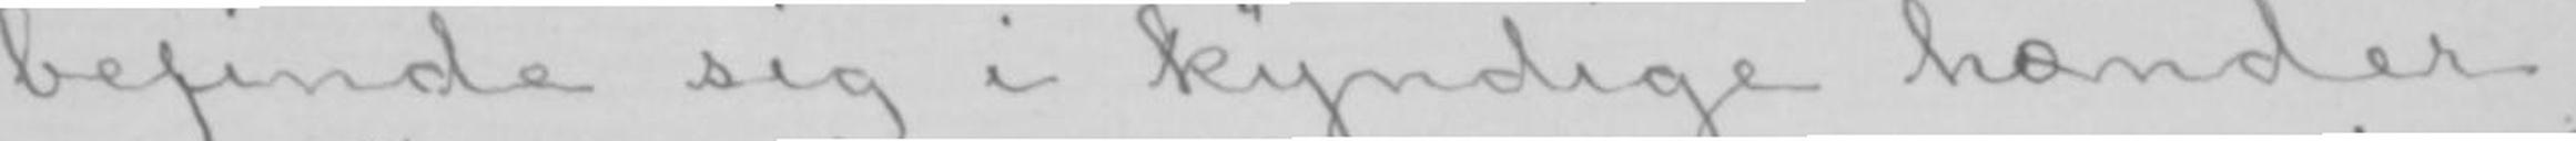befinde sig i kyndige hænder.
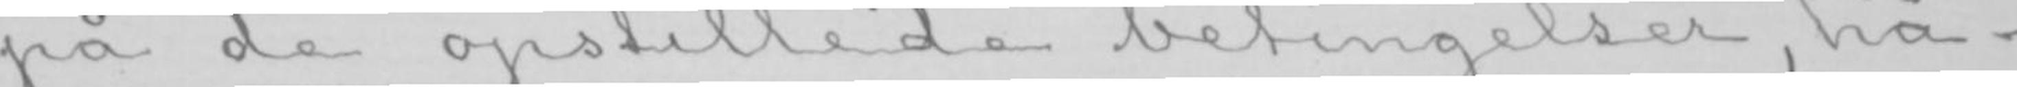på de opstillede betingelser, hå-
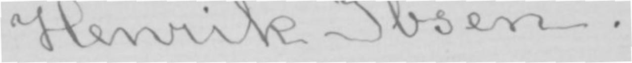Henrik Ibsen.
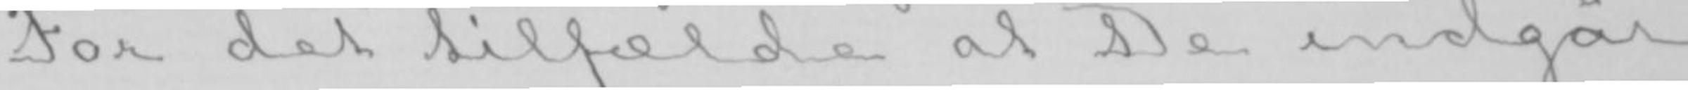For det tilfælde at De indgår
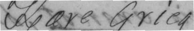Kjære Grieg
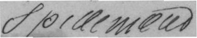Spillemænd
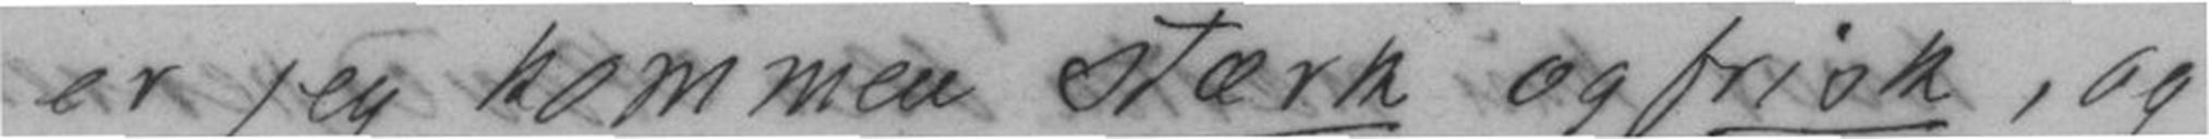er jeg kommen stærk og frisk, og
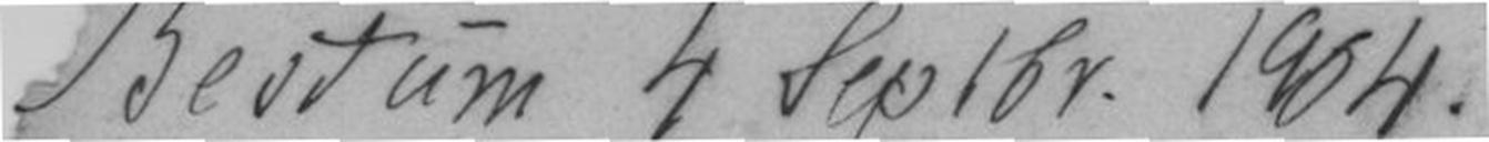Bestum 4 Septbr. 1904
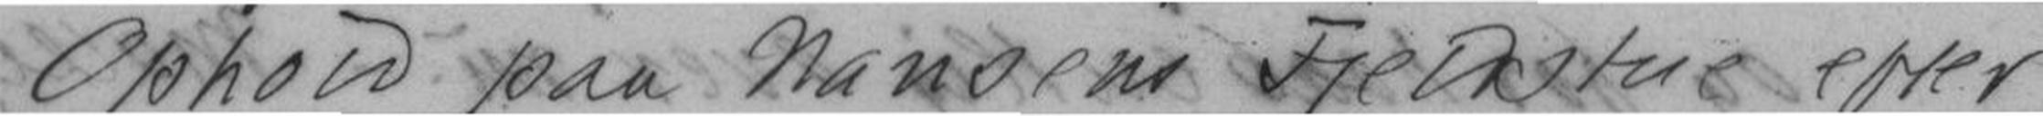Ophold paa Nansens Fjelstue efter
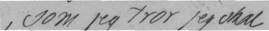, som jeg tror jeg skal
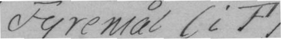Fyremål (i P)
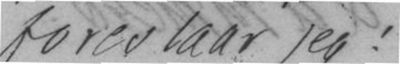foreslaar jeg:
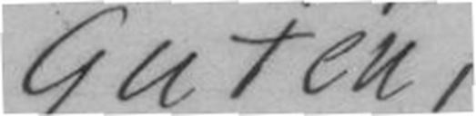Guten,
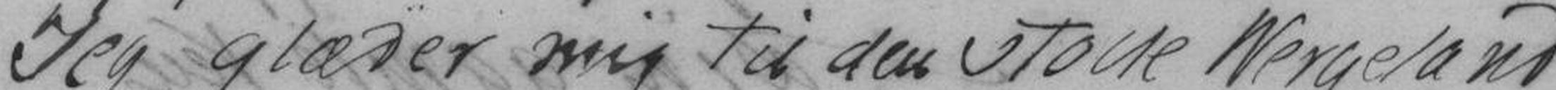Jeg glæder mig til den stolte Wergeland
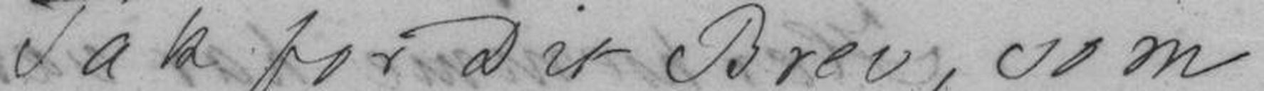Tak for Dit Brev, som
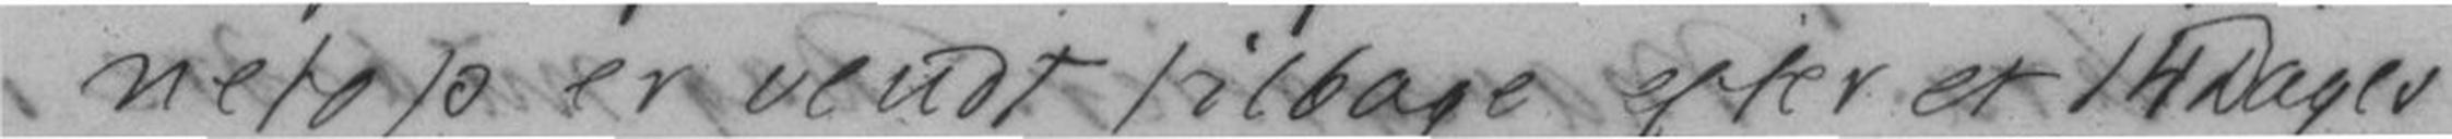netop er vendt tilbage efter et 14 Dagers
